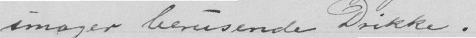smager berusende Drikke.
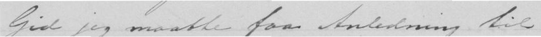Gid jeg maatte faa Anledning til
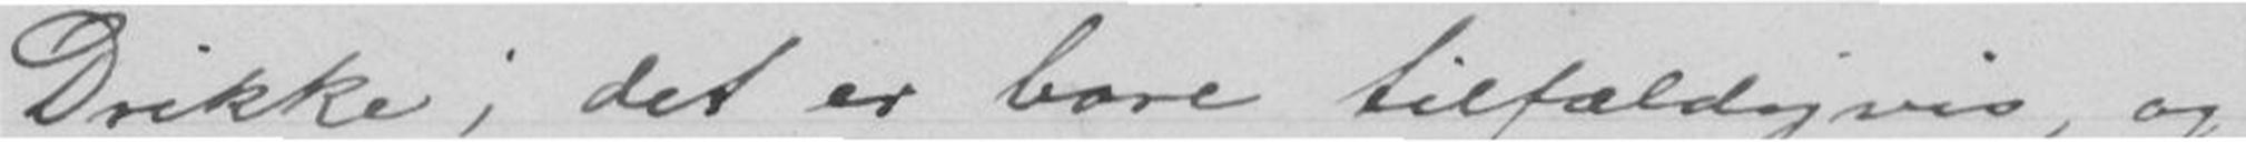Drikke; det er bare tilfældigvis, og
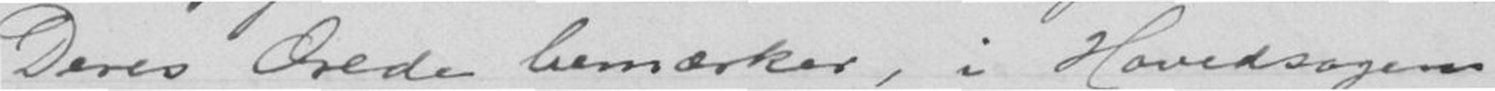Deres Ærede bemærker, i Hovedsagen
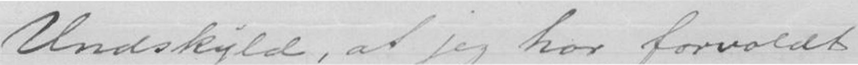Undskijld, at jeg har forvoldt
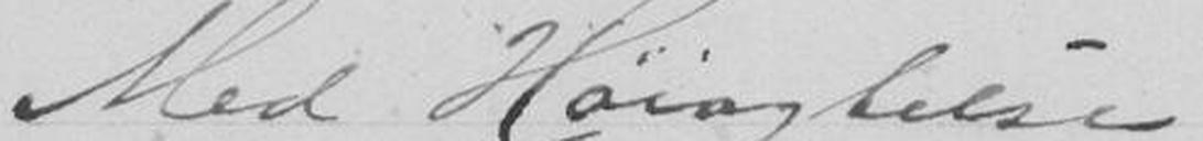Med Høiagtelse
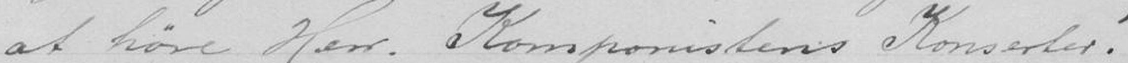at høre Herr Komponistens Konserter !
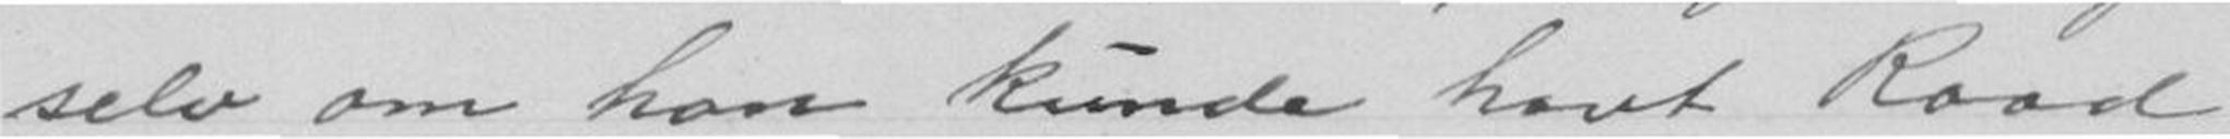selv om han kunde havt Raad
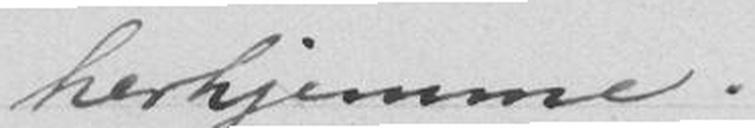herhjemme.
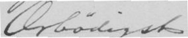Ærbødigst
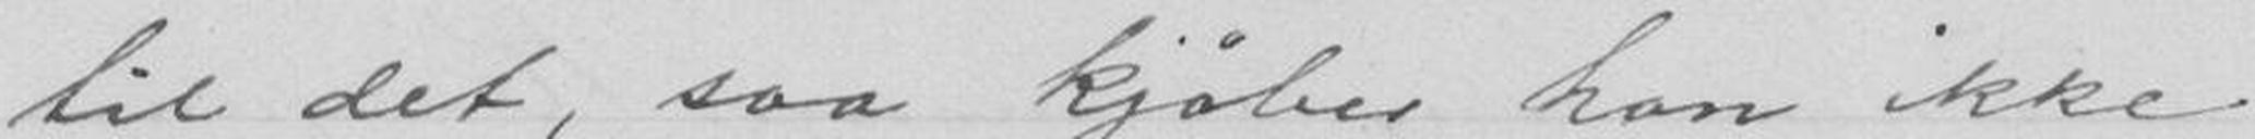til det, saa kjøber han ikke
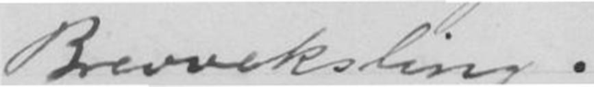Brevveksling.
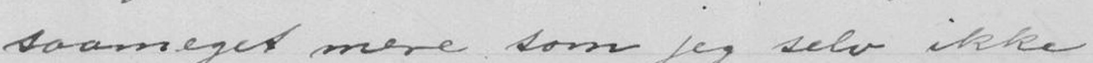saameget mer som jeg selv ikke
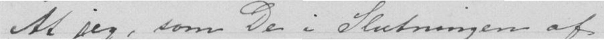At jeg, som De i Slutningen af
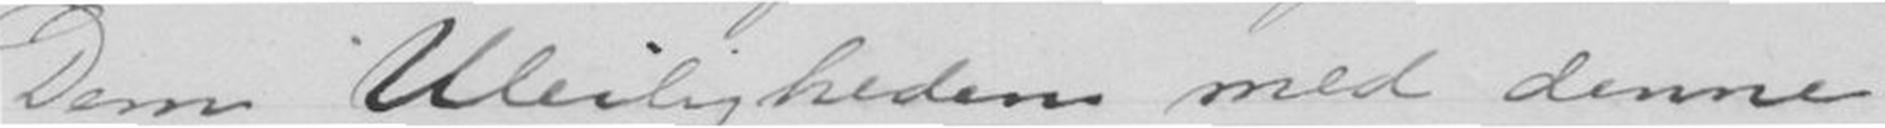Dem Uleiligheden med denne
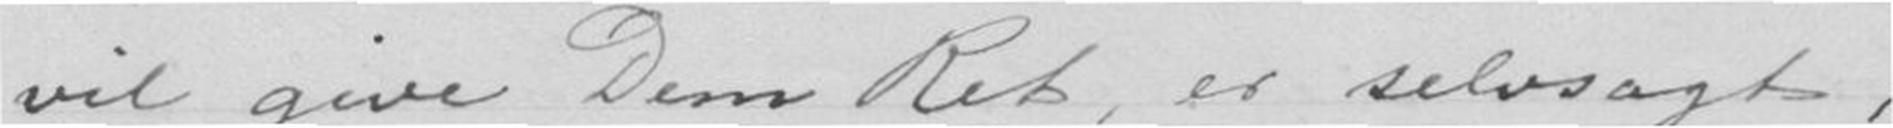vil give Dem Ret, er selvsagt,
